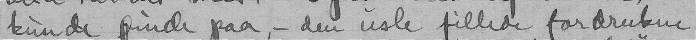kunde finde paa, - den usle fillede fordrukne
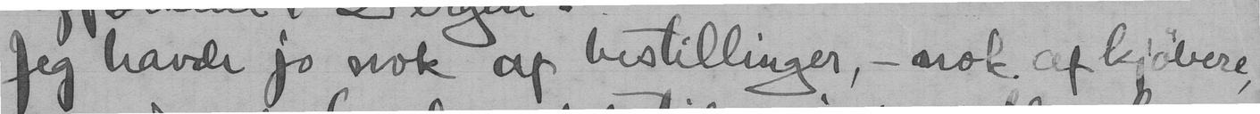Jeg havde jo nok af bestillinger, - nok af kjøbere
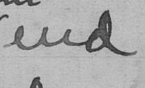end
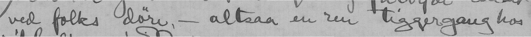ved folks døre, - altsaa en ren tiggergang hos
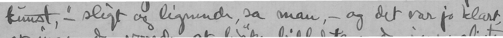kunst," - sligt og lignende, sa man, - og det var jo klart,
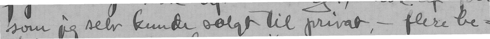som jeg selv kunde solgt til privat - flere be-
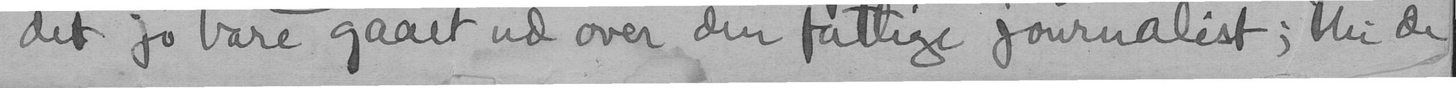det jo bare gaaet ud over den fattige journalist; thi de
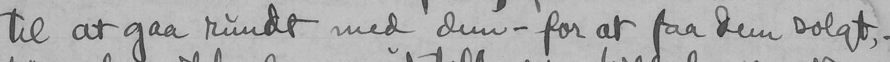til at gaa rundt med dem - for at faa dem solgt;-
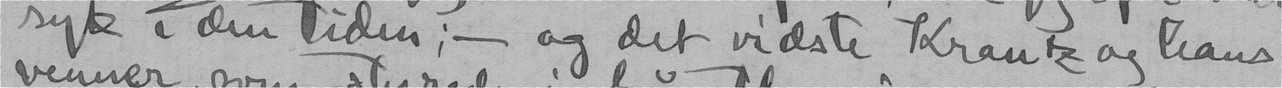syk i den tiden; - og det vidste Krantz og hans
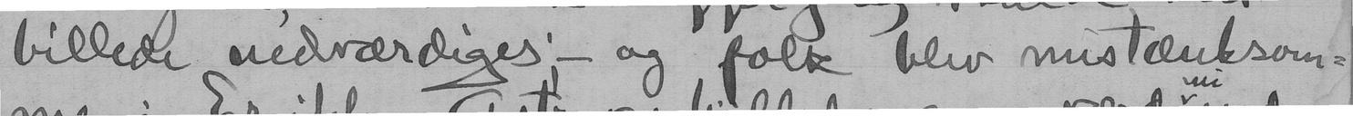billede nedværdiges - og folk blev mistænksom-
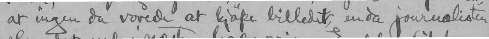at ingen da vovede at kjøpe billedet enda journalisten
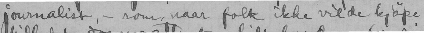journalist, - som, naar folk ikke vilde kjøpe
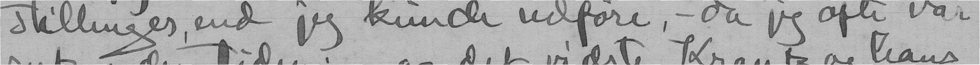stillinger end jeg kunde udføre, - da jeg ofte var
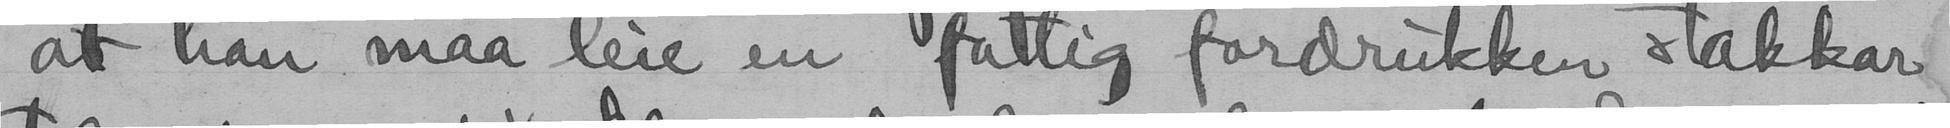at han maa leie en fattig fordrukken stakkar
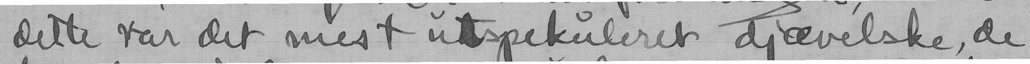dette var det mest udspekuleret djævelske, de
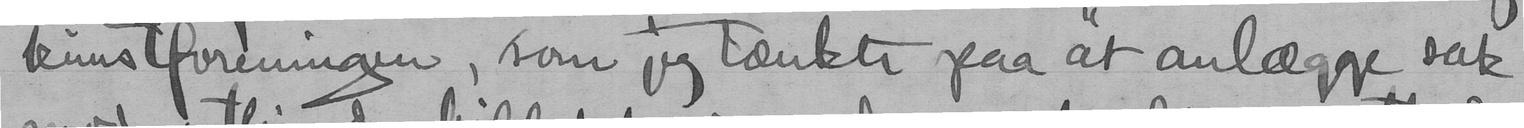kunstføreningen, som jeg tænkte paa at anlægge sak
